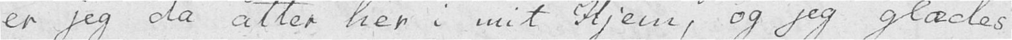er jeg da atter her i mit Hjem, og jeg glædes
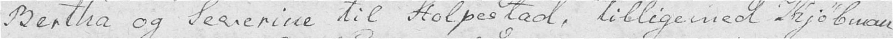Bertha og Severine til Stolpestad, tilligemed Kjøbman
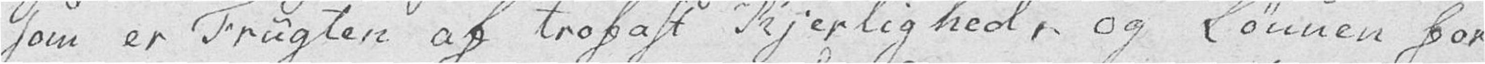som er Frugten af trofast Kjerlighed, og Lønnen for
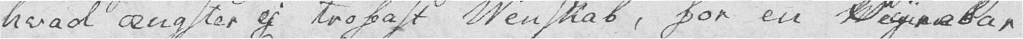hvad ængster ej trofast Venskab, for en Dyrebar
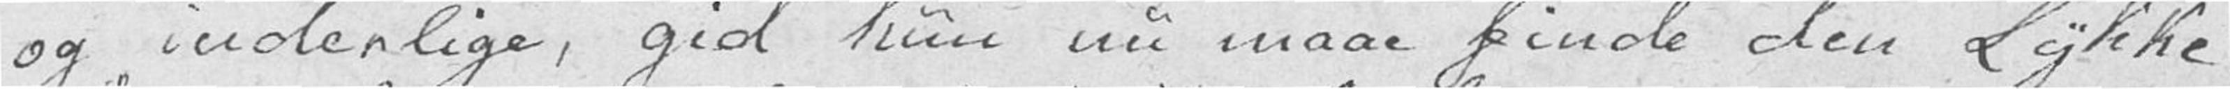og inderlige, gid hun nu maae finde den Lykke
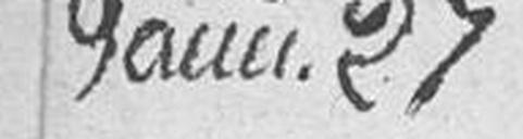Janu. 27
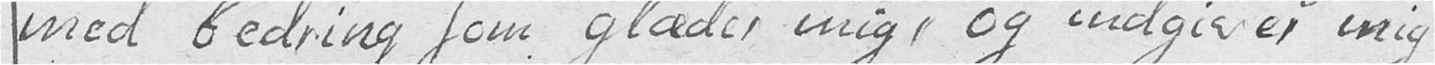med bedring som glæder mig, og indgiver mig
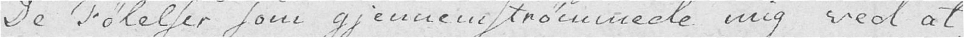De Følelser som gjennemstrømmede mig ved at
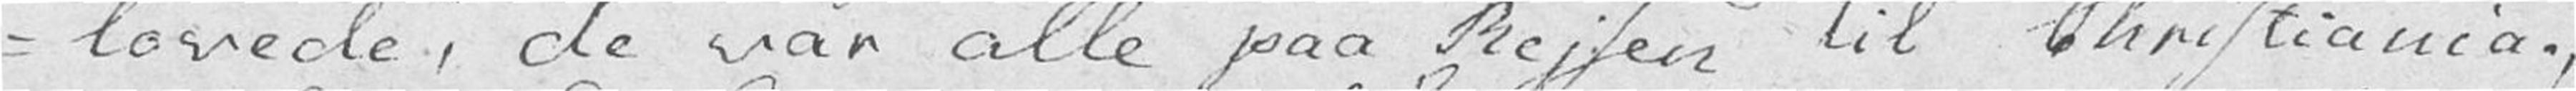-lovede, de var alle paa Rejsen til Christiania.
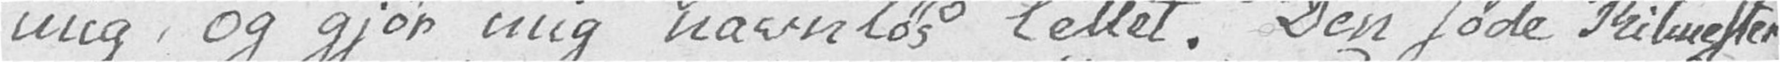mig, og gjør mig navnløs lettet. Den søde Ritmester
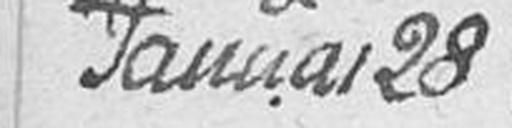Januar 28
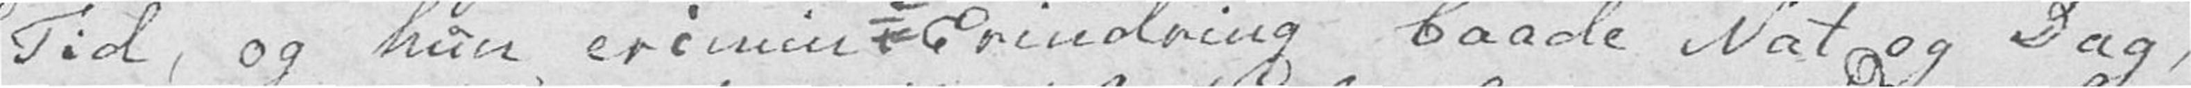Tid, og hun er i min i Erindring baade Nat og Dag,
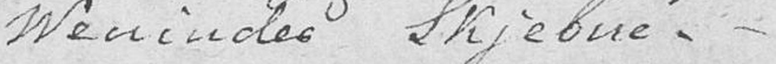Veninde Skjebne. –
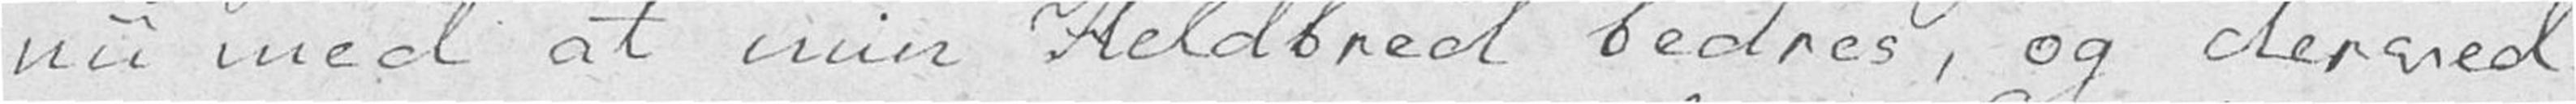nu med at min Heldbred bedres, og derved
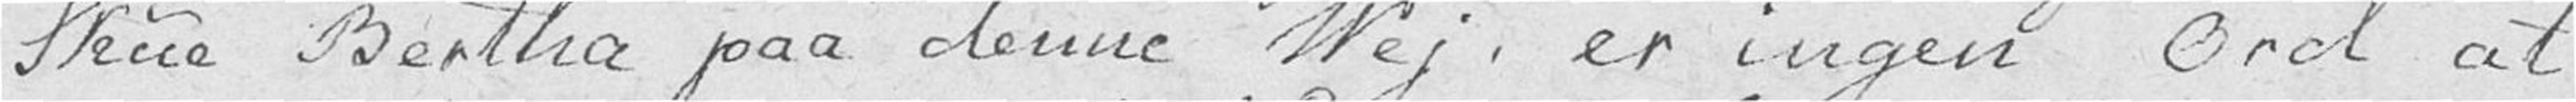Skue Bertha paa denne Vej, er ingen Ord at
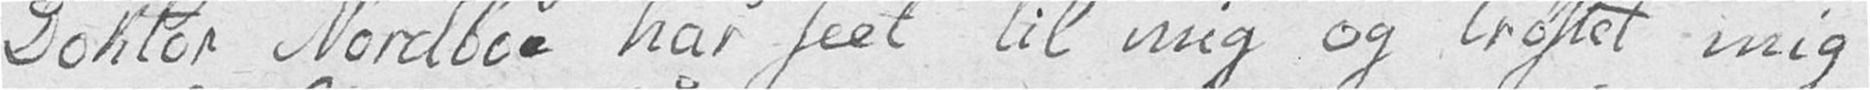Doktor Nordboe har seet til mig og trøstet mig
